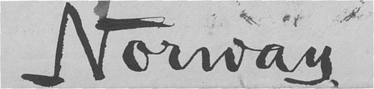Norway.
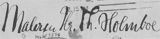Maleren Hr. Th. Holmboe
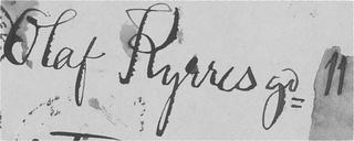Olaf Kyrres gd 11
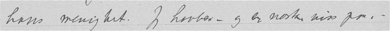hans menighet. Jeg haaber – og er næsten viss paa, –
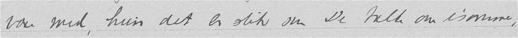være med, hvis det er slik som De talte om isommer,
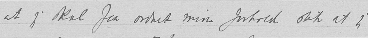at jeg skal faa ordnet mine forhold slik at jeg
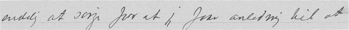endelig at sørge for at jeg faar anledning til at
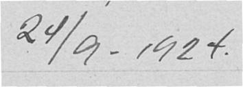24/9- 1924.
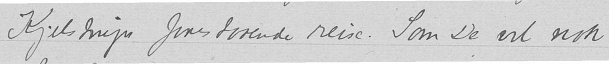Kjelstrups forestaaende reise. Som De vel nok
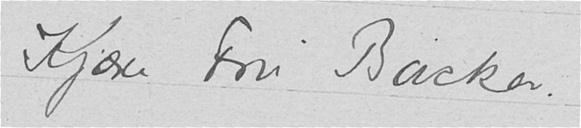Kjære Fru Backer.
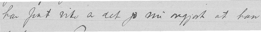har faat vite er det jo nu avgjort at han
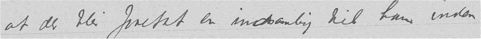at der blir foretat en indsamlig til ham inden
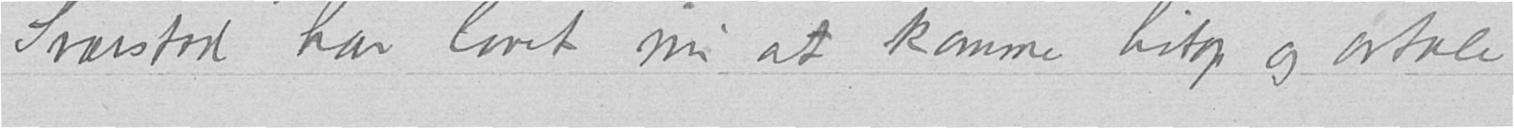Svarstad har lovet nu at komme hitop og avtale
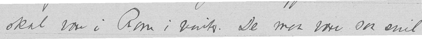skal være i Rom i vinter. De maa være saa snil
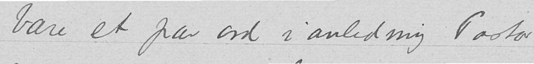bare et par ord i anledning Pastor
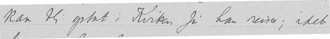kan bli optat i Kirken før han reiser; idet
